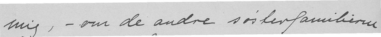mig, - om de andre søsterfamilierne
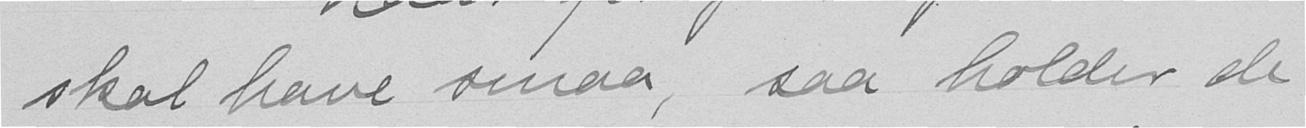skal have smaa, saa holder de
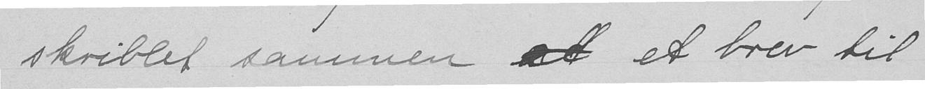skriblet sammen at et brev til
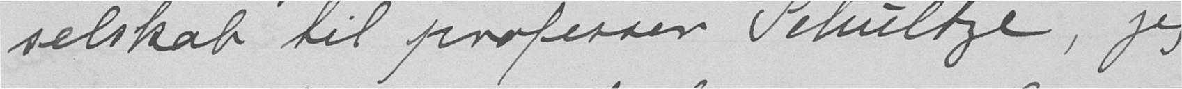selskab til professor Schultze, jeg
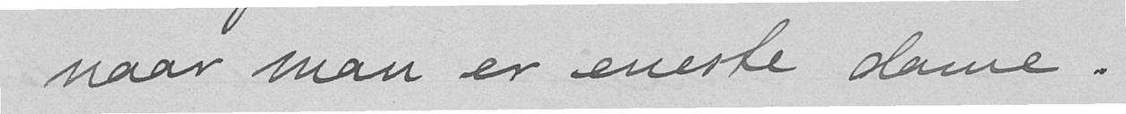naar man er eneste dame.
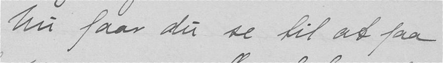Nu faar du se til at faa
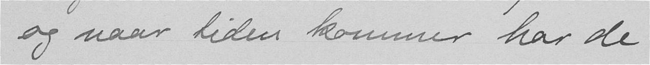og naar tiden kommer har de
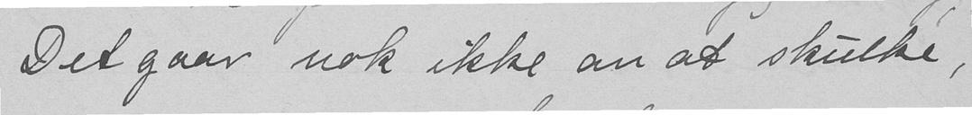Det gaar nok ikke an at skulke,
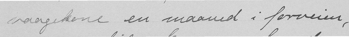vaagekone en maaned i forveien,
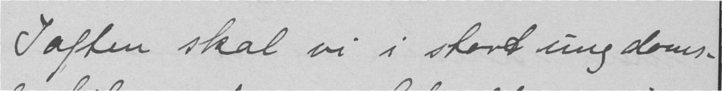I aften skal vi i stort ungdoms-
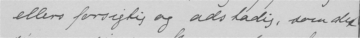forsigtig og adstadig, som det
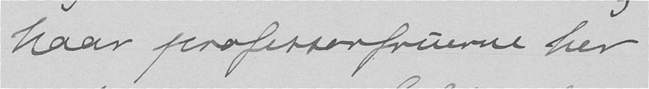Naar professorfruerne her
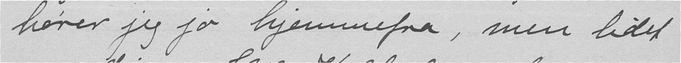hører jeg jo hjemmefra, men lidet
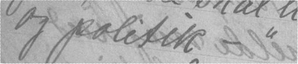og politik - "
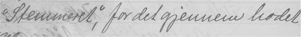"Stemmeret", for det gjennem hodet
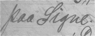paa Signe.
